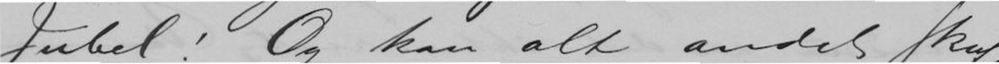Jubel! Og kan alt andet skuffe
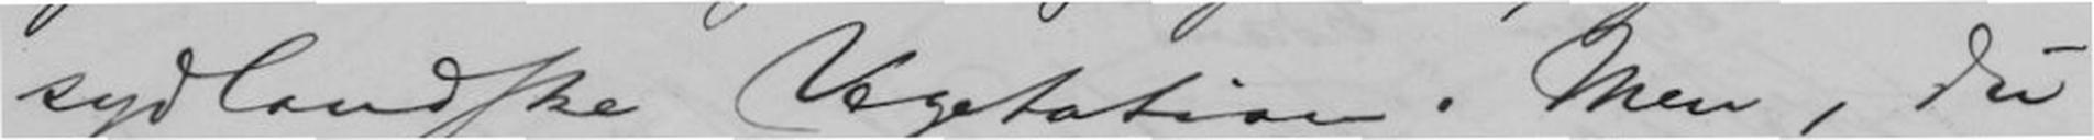sydlandske Vegetation. Men, Du
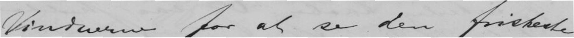Vinduerne for at se den friskeste
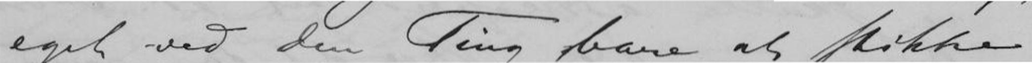eget ved den Ting bare at stikke
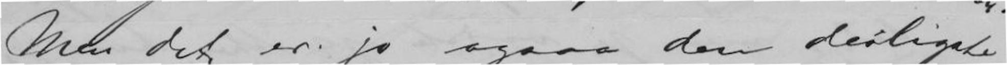Men det er jo ogsaa den deiligste
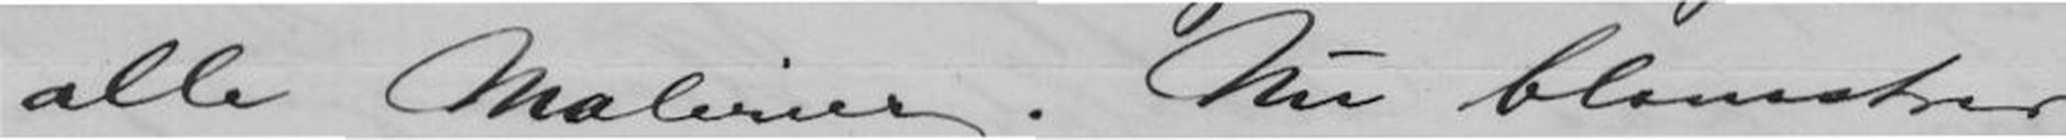alle Malerier. Nu blomstrer
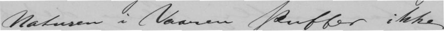- Naturen i Vaaren skuffer ikke.
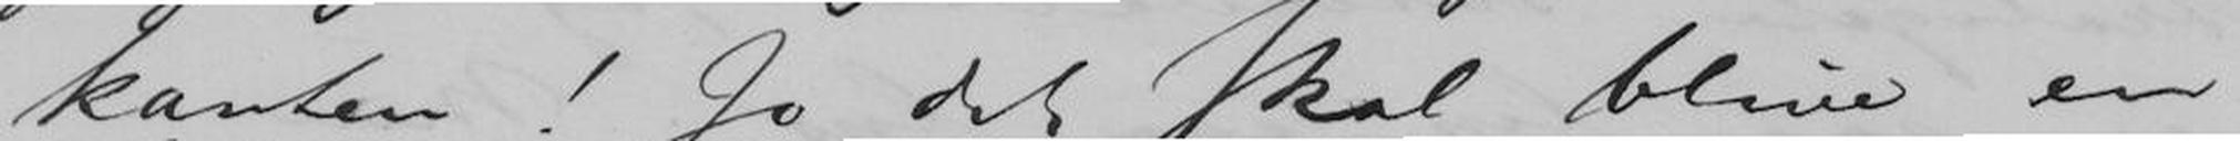kanten! Jo det skal blive en
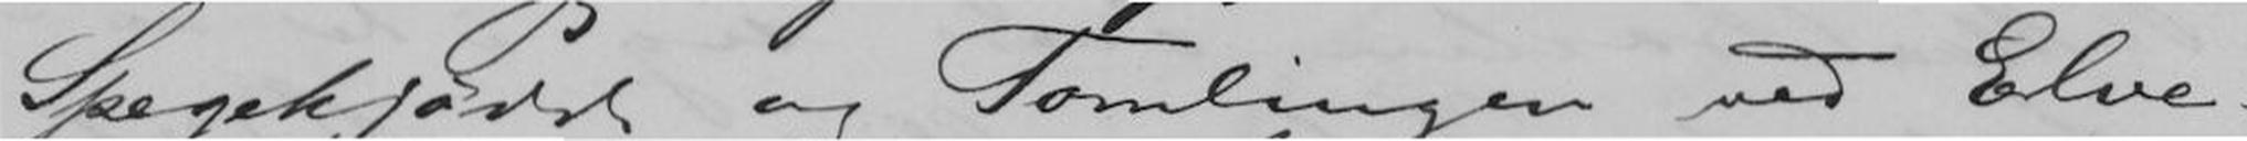Spegekjødet og Tomlingen ved Elve-
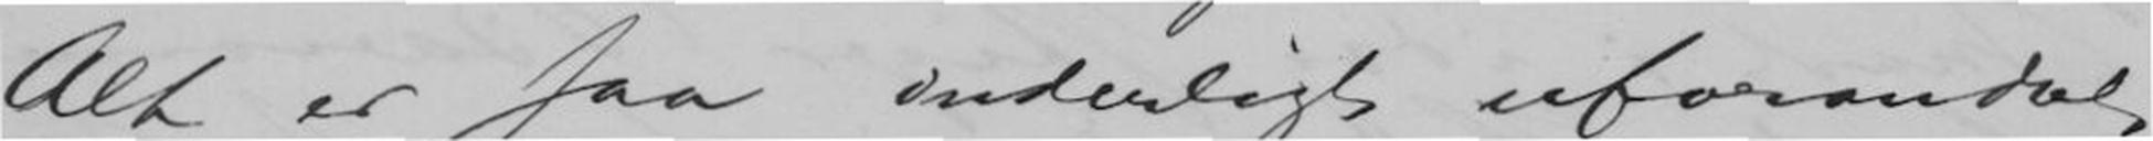Alt er saa inderligt uforandret.
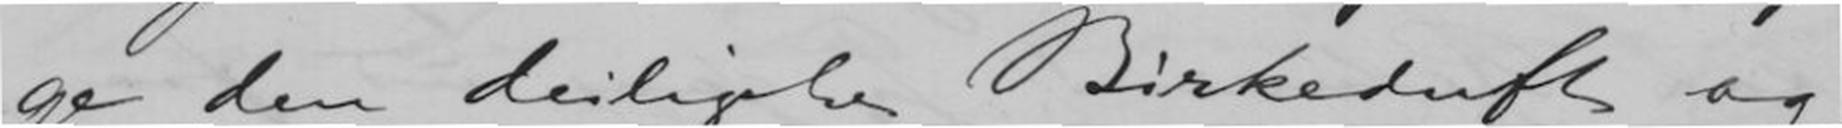ge den deiligste Birkeduft og
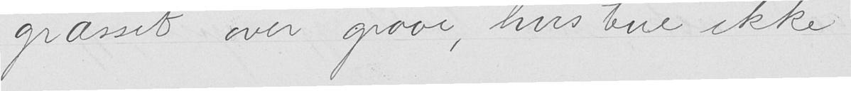græsset over grave, hvis tue ikke
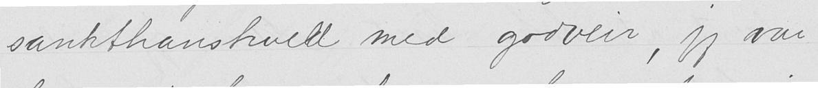sankthanskvell med godveir, jeg var
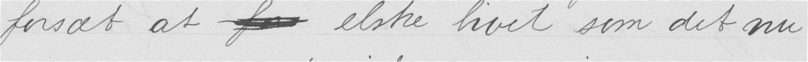forsæt at for elske livet som det nu
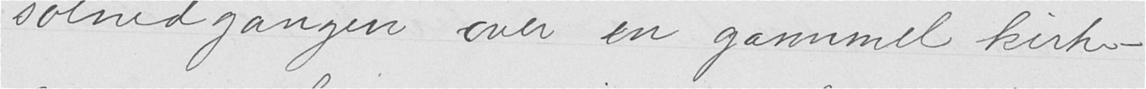solnedgangen over en gammel kirke-
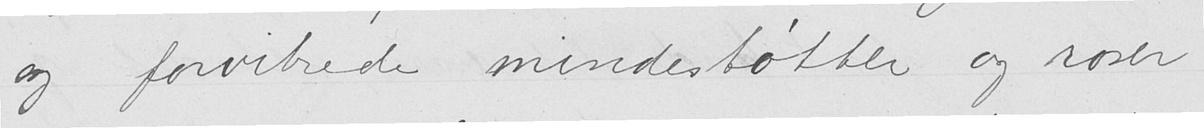og forvitrede mindestøtter og roser
